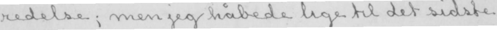redelse; men jeg håbede lige til det sidste
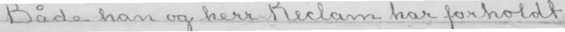Både han og herr Reclam har forholdt
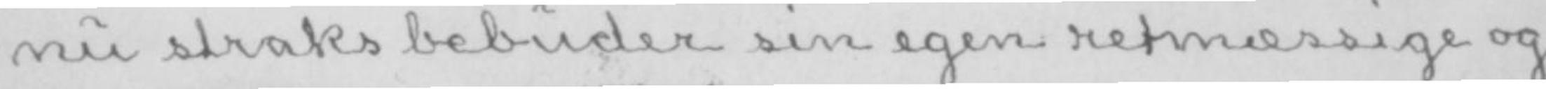nu straks bebuder sin egen retmæssige og
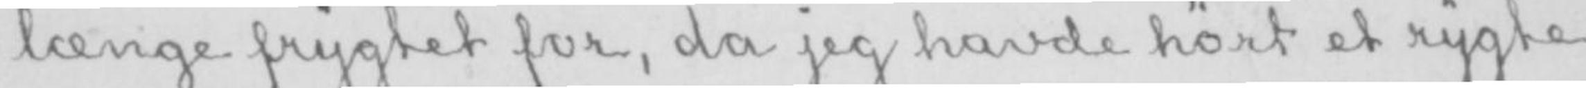længe frygtet for, da jeg havde hørt et rygte
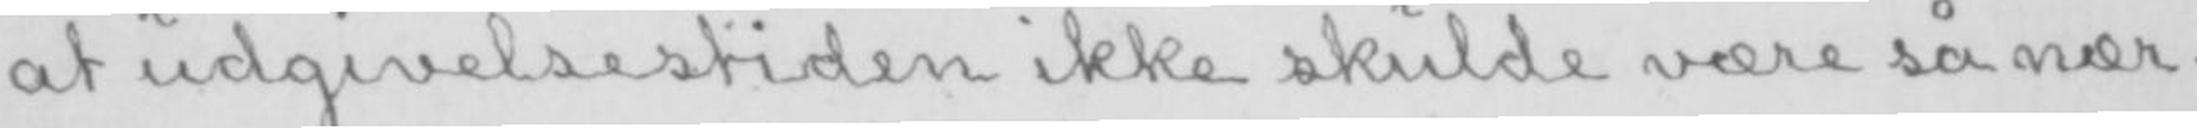at udgivelsestiden ikke skulde være så nær.
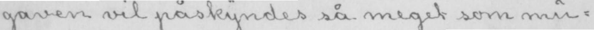gaven vil påskyndes så meget som mu-
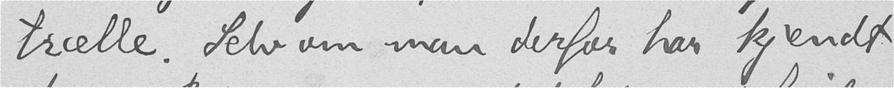trælle. Selv om man derfor har kjendt
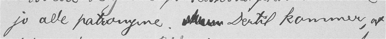jo alle patronyme.  Dertil kommer, at
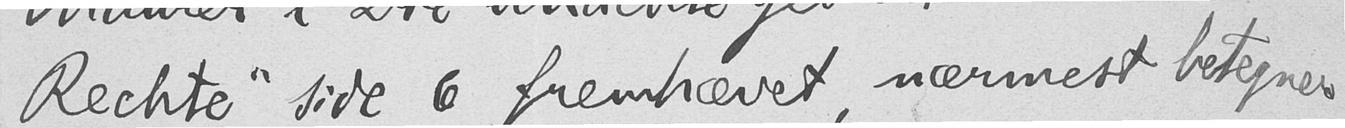Rechte" side 6 fremhævet, nærmest betegner
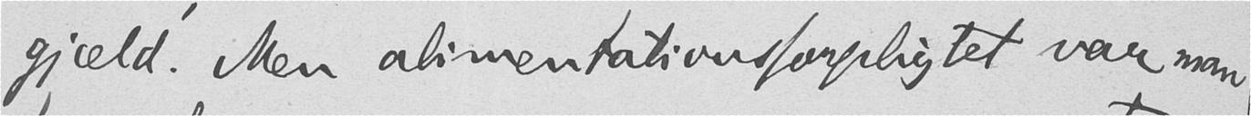gjæld. Men alimentationsforpligtet var man
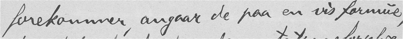forekommer, angaar de paa en vis formue,
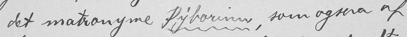det matronyme þýborinn, som ogsaa af
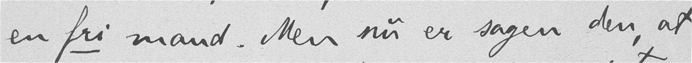en fri mand. Men nu er sagen den, at
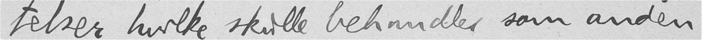telser, hvilke skulle behandles som anden
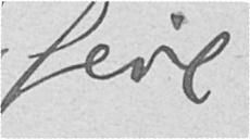feil
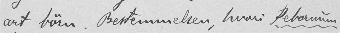art børn. Bestemmelsen, hvori þeborinn
